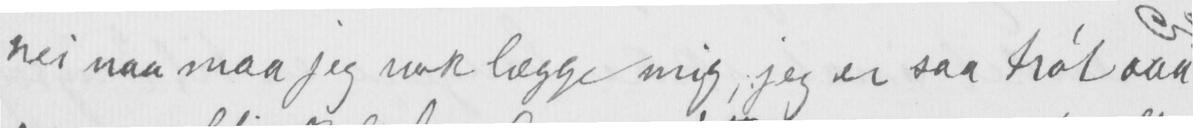Nei naa maa jeg nok lægge mig, jeg er saa trøt saa
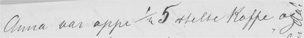Anna var oppe ½ 5 stelte Kaffe og
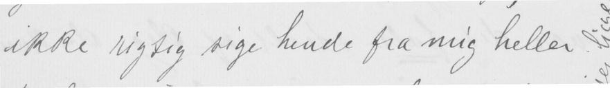ikke rigtig sige hende fra mig heller
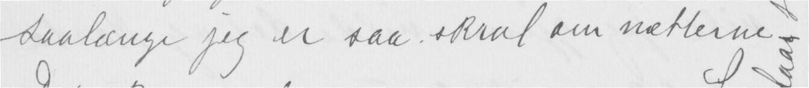– saalænge jeg er saa skral om netterne –
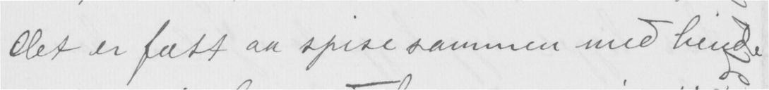det er fælt aa spise sammen med hende
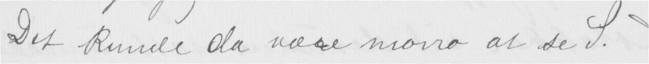Det kunde da være morro at se S.
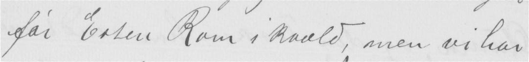før Ester Ram i kvæld, men vi har
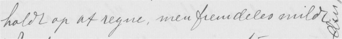holdt op at regne, men fremdeles mildt.
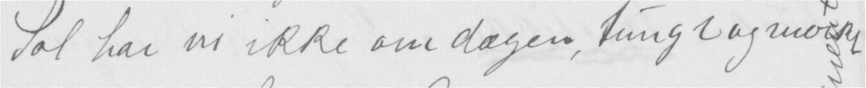Sol har vi ikke om dagen, tungt og mørkt
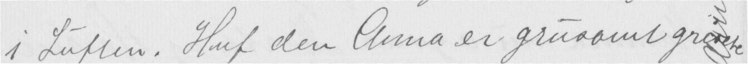i Luften. Huf den Anna er grusomt grisete
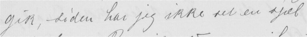gik, siden har jeg ikke set en sjæl
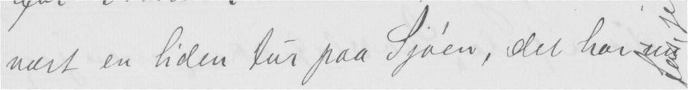vært en liden tur paa Sjøen, det har nu
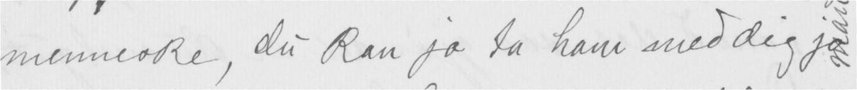menneske, du kan jo ta ham med dig jo
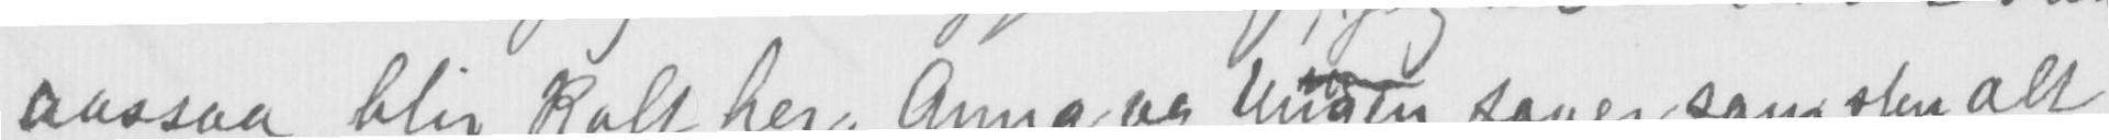aassaa blir kalt her. Anna og Ungen sover som sten alt
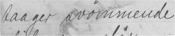taager svømmende
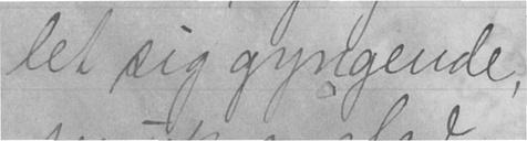let sig gyngende,
